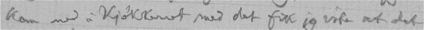kom ned i Kjøkkenet med det fik jeg vite at det
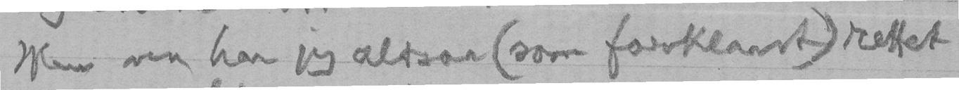Men nu har jeg altsaa (som forklaret) rettet
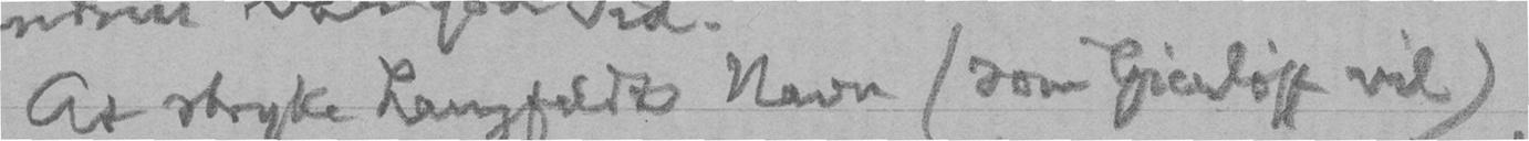At stryke Langfeldts Navn (som Gierløff vil)
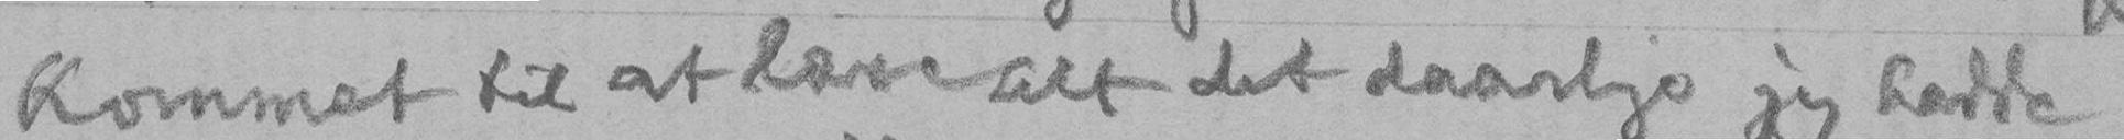kommet til at læse alt det daarlige jeg hadde
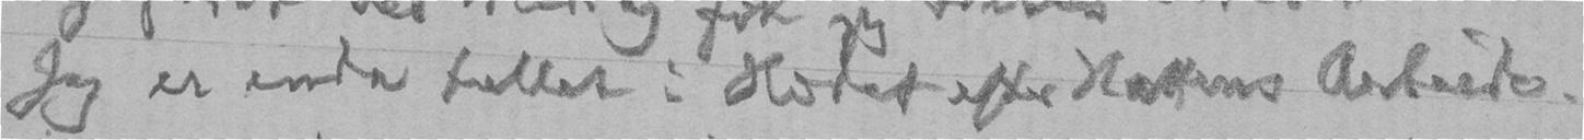Jeg er enda tullet i Hodet efter Nattens Arbeide.
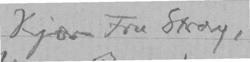Kjære Fru Stray,
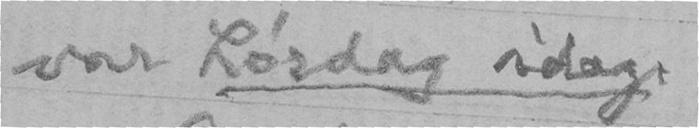var Lørdag idag.
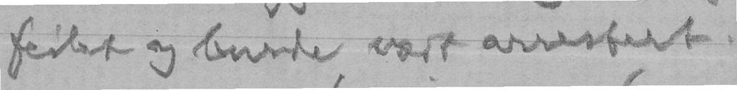feilet og burde vært arrestert.
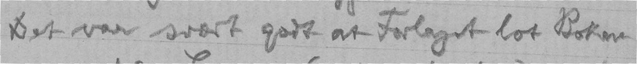Det var svært godt at Forlaget lot Boken
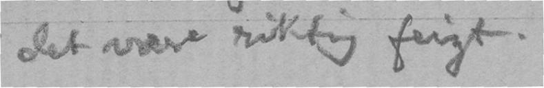det være riktig feigt.
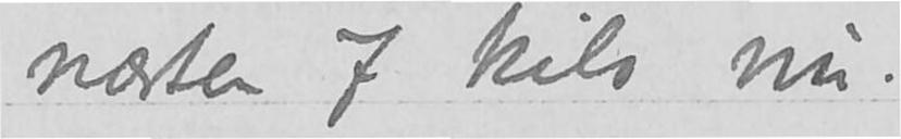næsten 7 kilo nu.
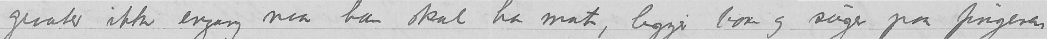graater ikke engang naar han skal ha mat, ligger bare og suger paa fingeren
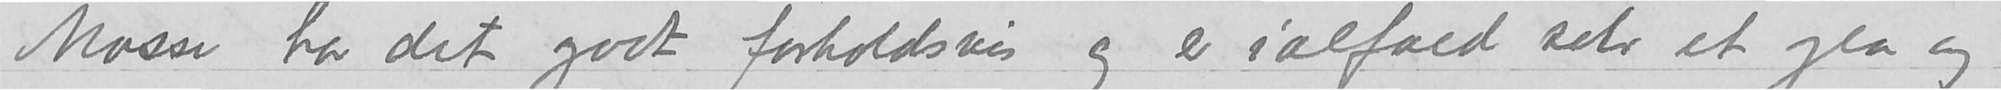Mosse har det godt forholdsvis og er ialfald selv et gla og
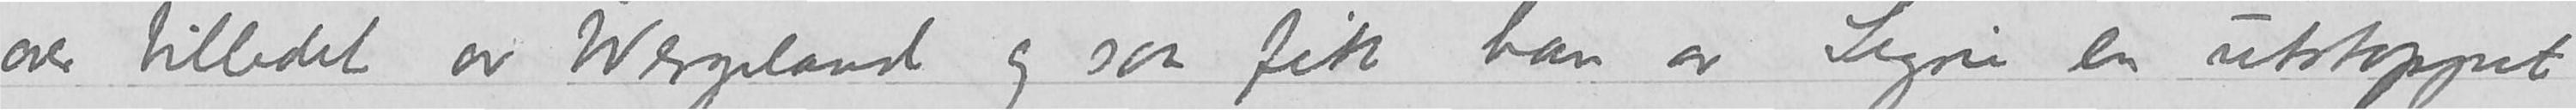over billedet av Wergeland og saa fik han av Signe en utstoppet
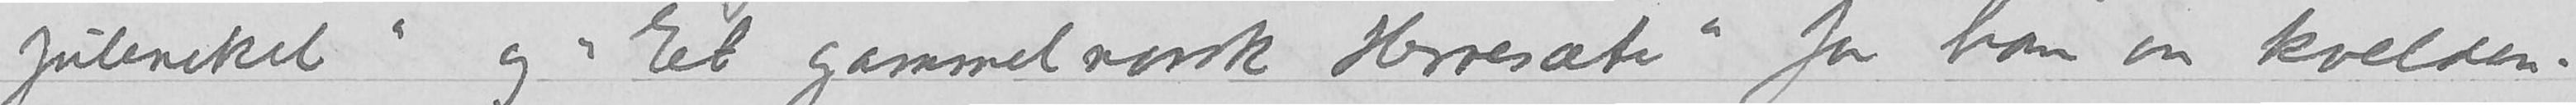juleneket» og «Eet gammelnorsk Herresæte» for ham om kvelden.
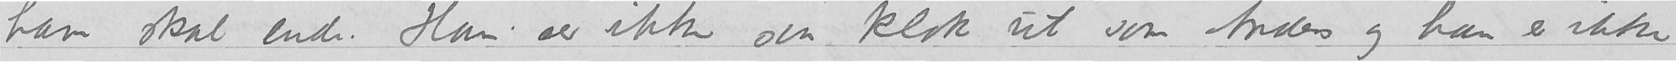ham skal ende.  Han ser ikke saa klok ut som Anders og han er ikke
